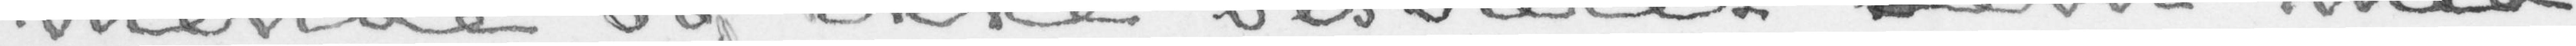mende og ikke besværet Dem med
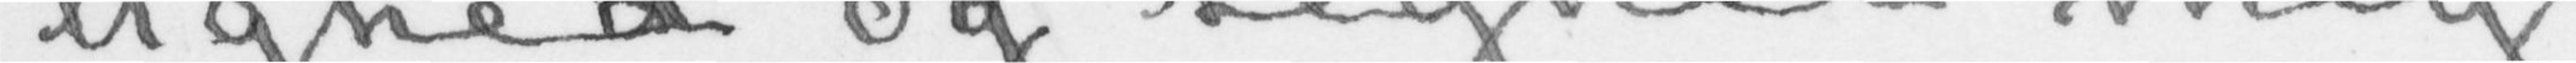lighed og tegner mig
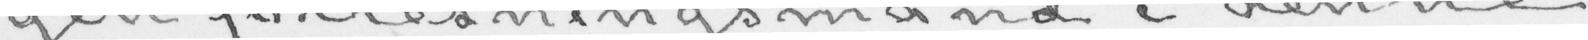gen forretningsmand i denne
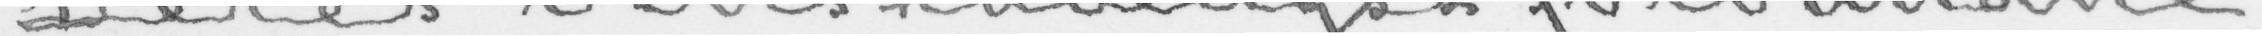Deres venskabeligst forbundne
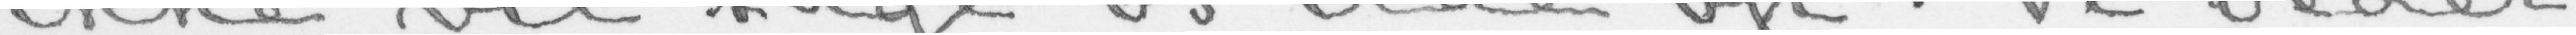ikke vil tage os ilde op. Vi beder
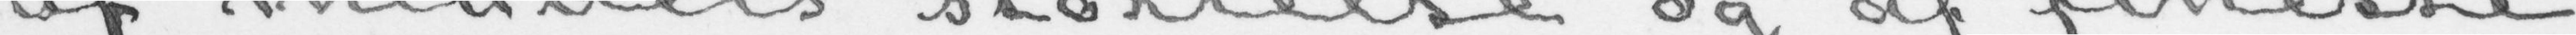af middels størrelse og af fineste
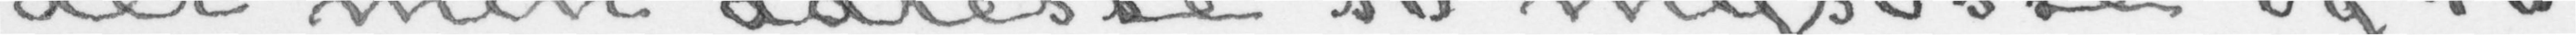der min adresse to mysoste og to
